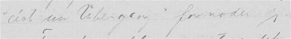"c'est un Übergang" formoder jeg.
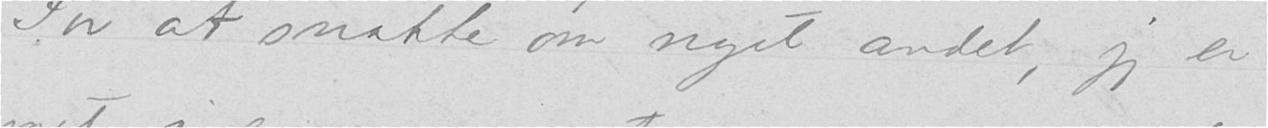For at snakke om noget andet, jeg er
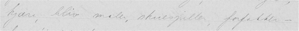kjære, bliv maler, skuespiller, forfatter —"
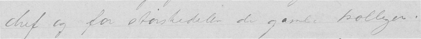chef og for størstedelen de gamle kolleger.
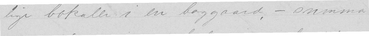lige lokaler i en baggaard, — summa
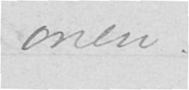onen.
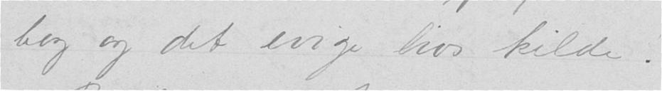bog og det evige livs kilde!
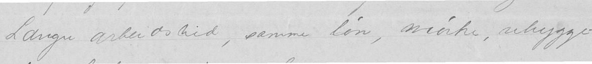Længre arbeidstid, samme løn, mørke, uhygge-
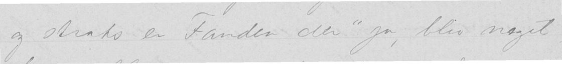og straks er Fanden der "Ja, bliv noget,
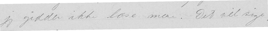jeg gidder ikke læse mere. Det vil sige,
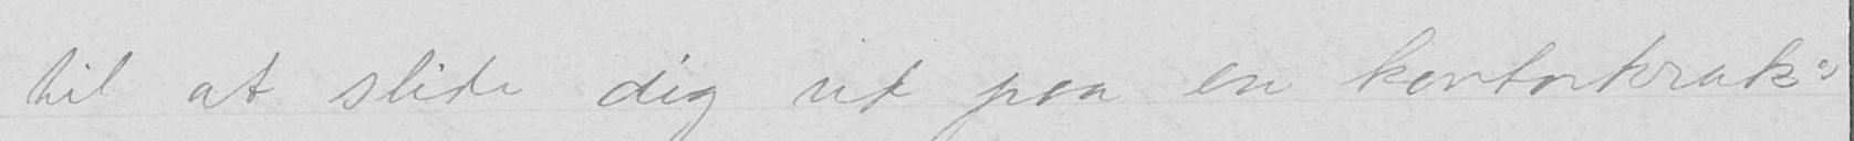til at slide dig ud paa en kontorkrak»
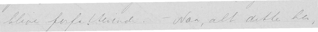blive forfatterinde. — Naa, alt dette her,
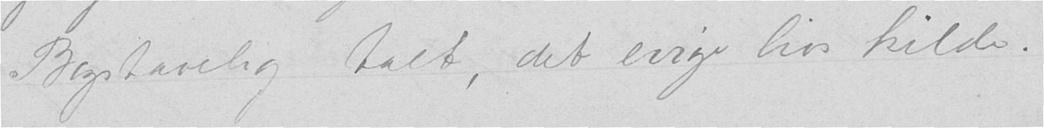Bogstavelig talt, det evige livs kilde.
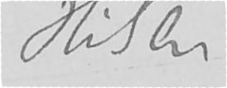Hilsen
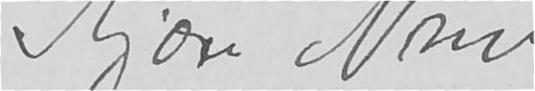Kjære Nini,
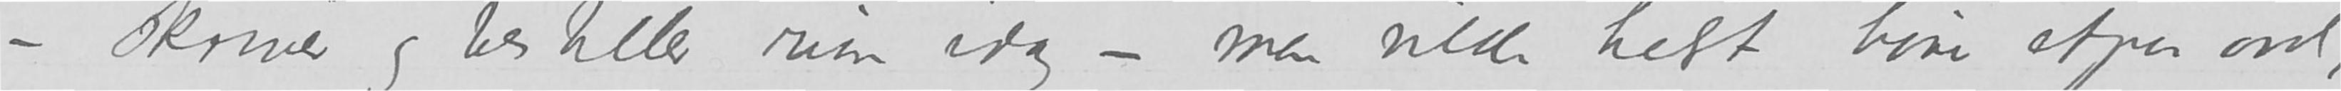– skriver og bestiller rum idag – men vilde helst høre etpar ord,
